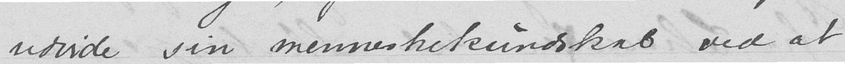udvide sin menneskekundskab ved at
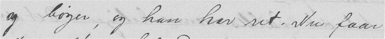og bøger, og han har ret. Nu faar
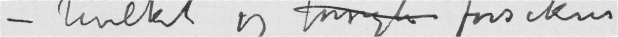– hvilket jeg forøvrigt forsikrer
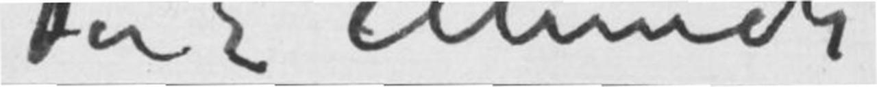Din E Munch
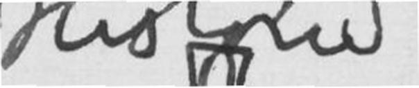Historie
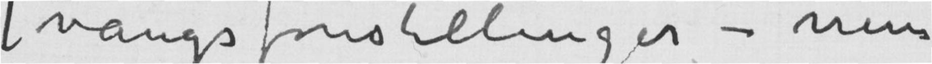Tvangsforestillinger – men
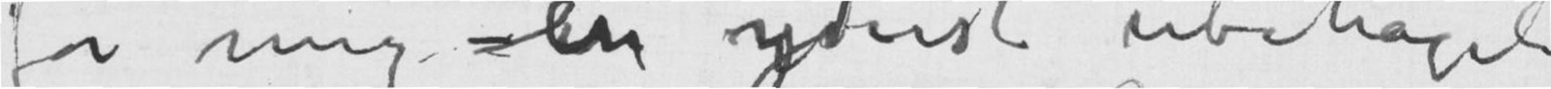for –mig en yderst ubehageli
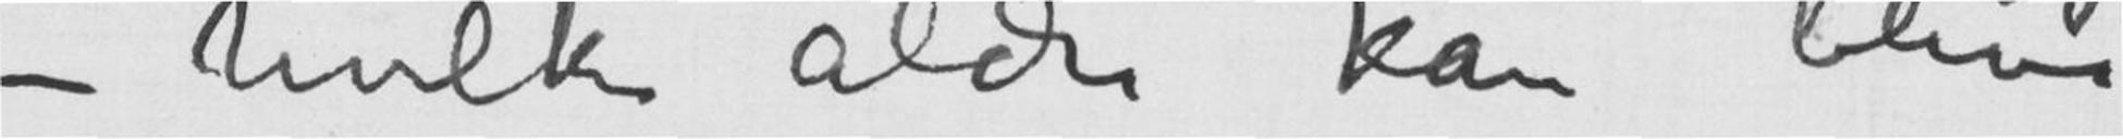– hvilke aldri kan blive
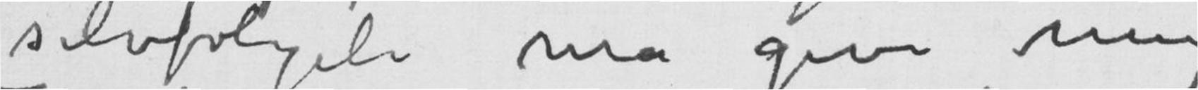selvfølgeli må give mig
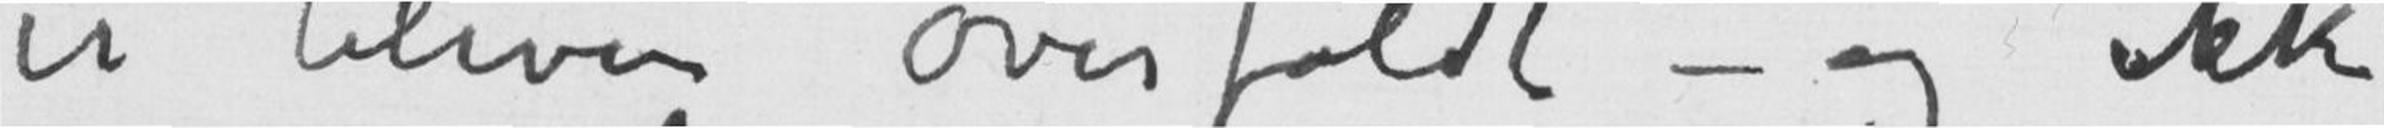er bleven overfaldt – og ikke
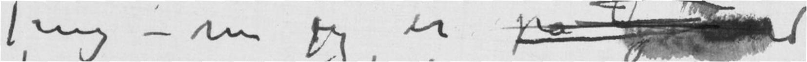Ting – nu jeg er på Grund
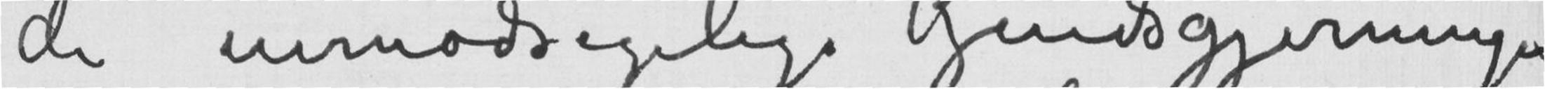de uimodsigelige Kjendsgjerninger
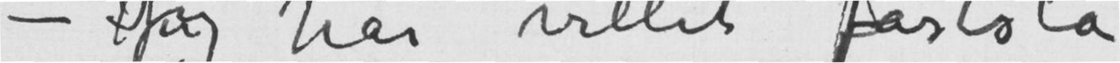– Jeg har villet fastslå
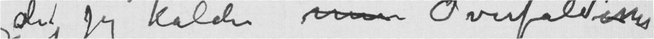det jeg kalder min Overfald-
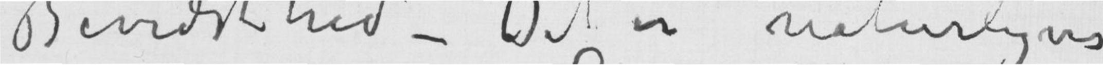Bevidshed – Det er naturligvis
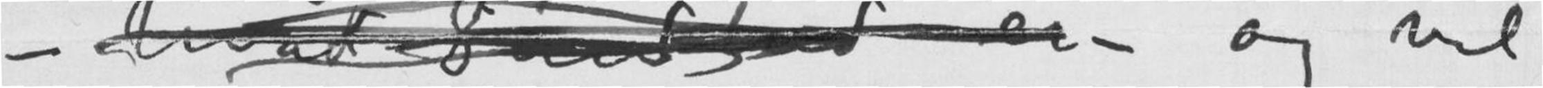– hvad– og vil
